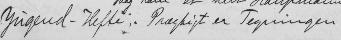Jugend-Hefte. Prægtigt er Tegningen
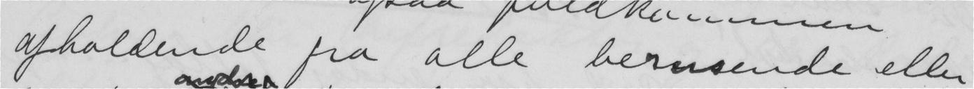Afholdende fra alle berusende eller
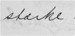stærke.
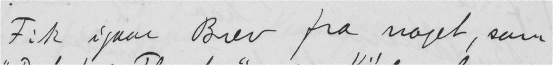Fik igaar Brev fra noget, som
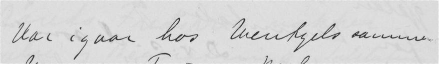Var igaar hos Wentzels sammen
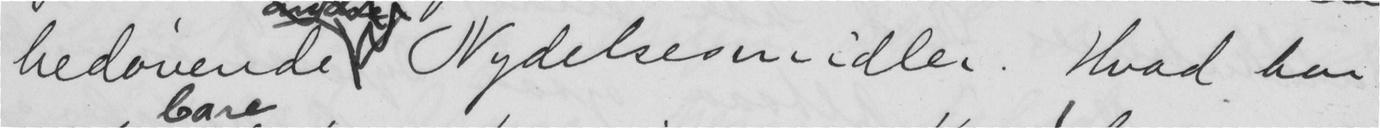bedøvende Nydelsesmidler. Hvad har
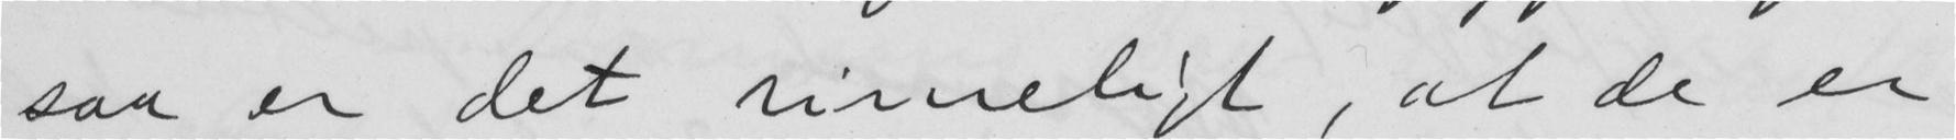saa er det rimeligt, at de er
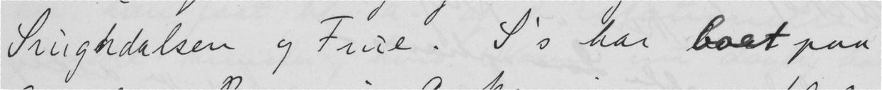Singhdalsen og Frue. S's har boet paa
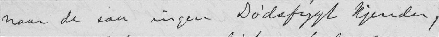naar de saa ingen Dødsfygt kjender,
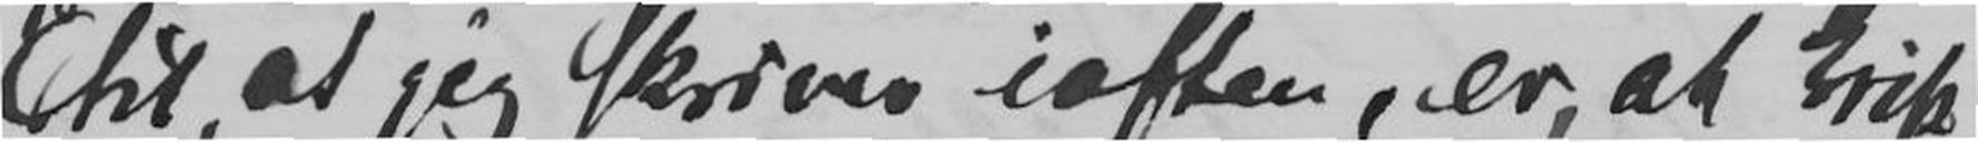til, at jeg skriver iaften, er, at Erik
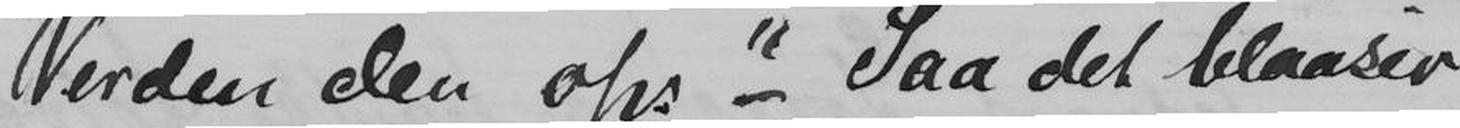Verden den osv." - Saa det blaaser
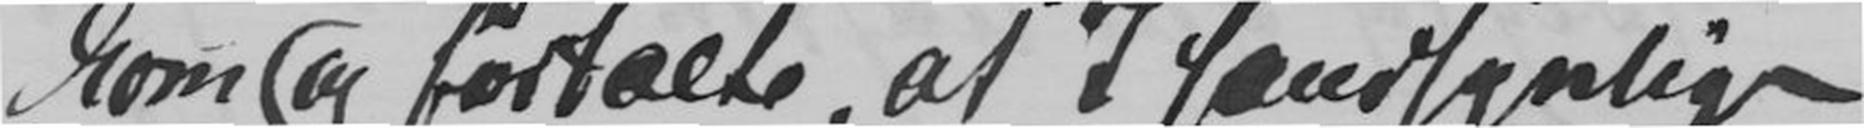kom og fortalte, at I sandsynlig
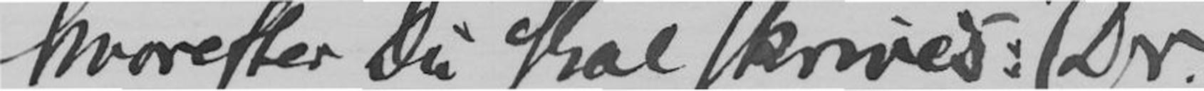hvorefter Du skal skrives "Dr.
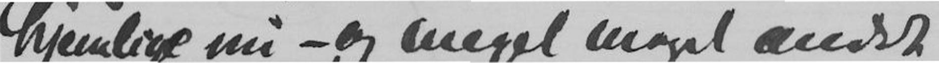hjemlige nu - og meget meget andet
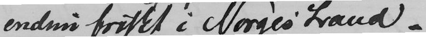endnu friskt i Norges Land.
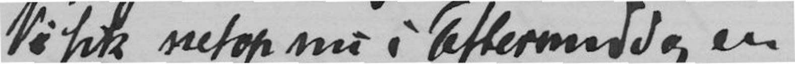Vi fik netop nu i Eftermiddag en
